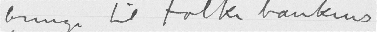bringe til Folkebankens
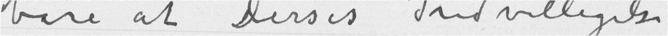bare at Deres Indvilligelse
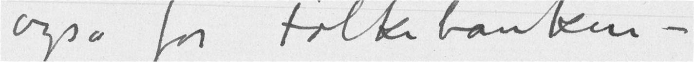også for Folkebanken –
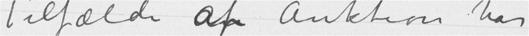Tilfælde af Auktion har
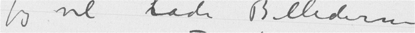jeg vil lade Billederne
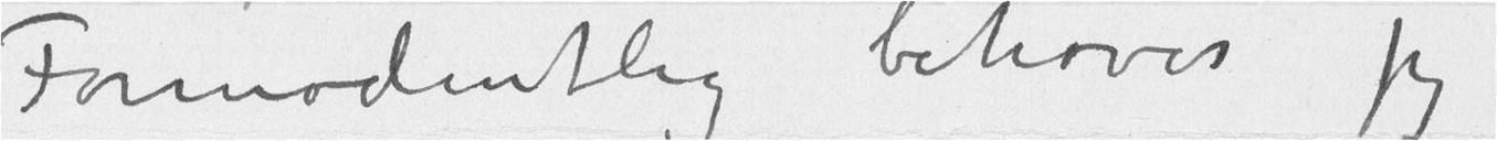Formodentlig behøver jeg
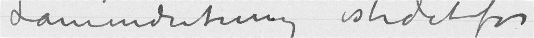Låneindretning istedetfor
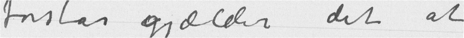forstår gjælder det at
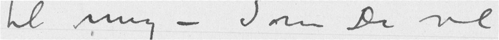til mig – Som De vel
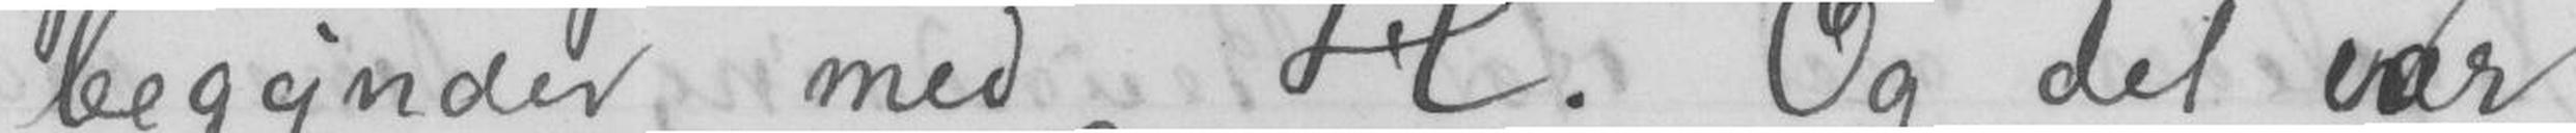begynder med H. Og det var
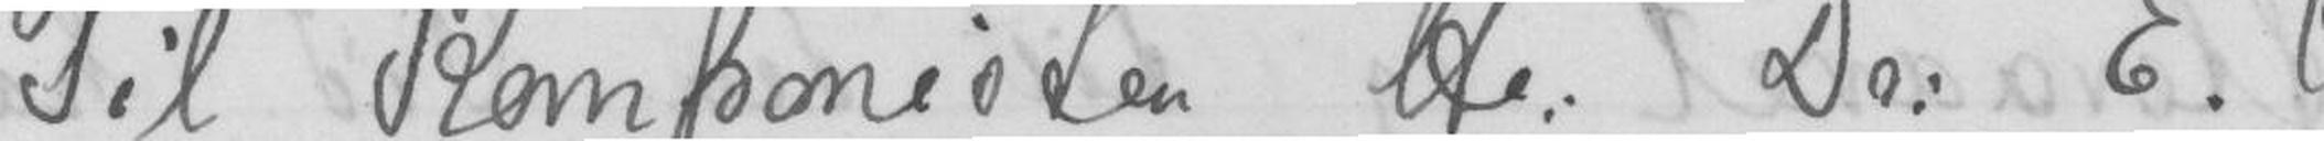Til Komponisten bl. Da: E.G.
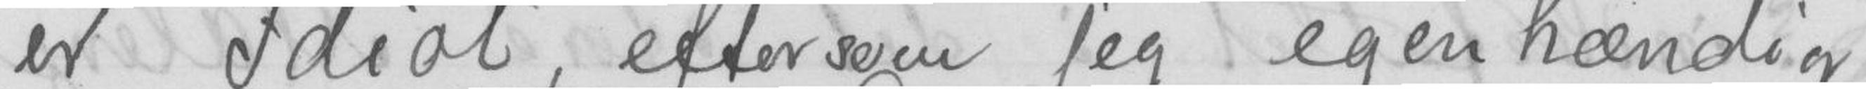er Idiot, eftersom jeg egenhændig
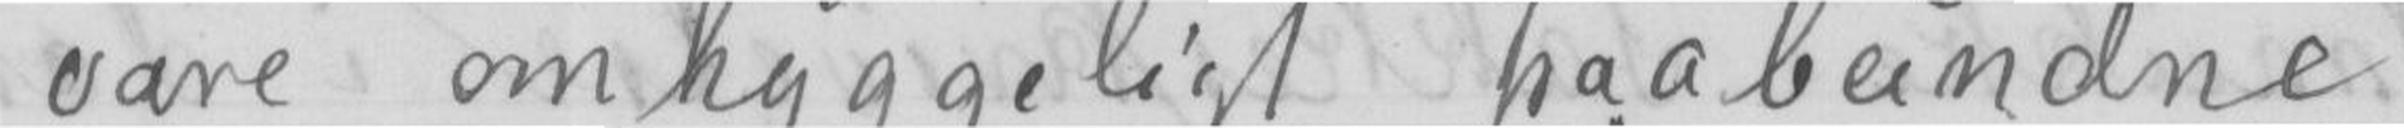være omhyggeligt paabundne
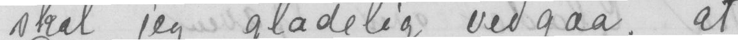skal jeg gladelig vedgaa, at
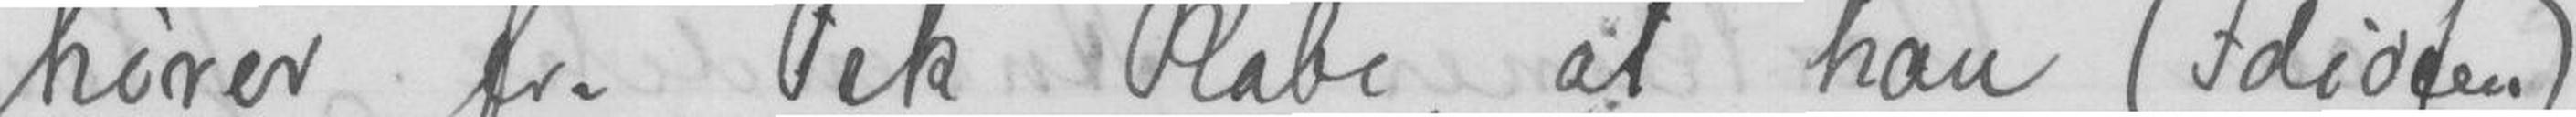hører fra Frk. Rabe at han (Idioten)
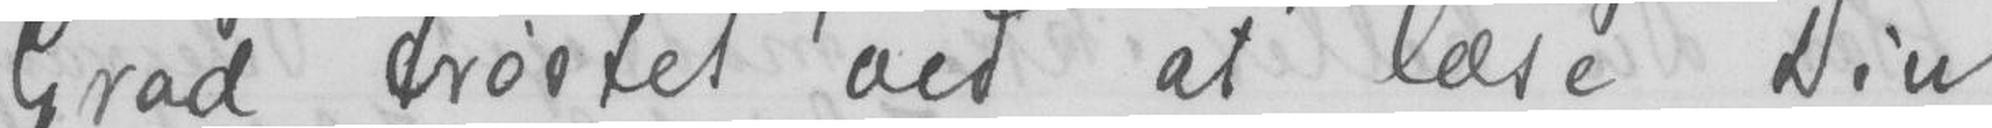Grad trøstet ved at læse Din
